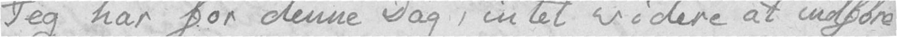Jeg har for denne Dag, intet videre at indføre
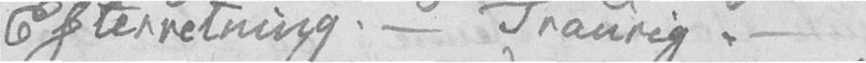Efterretning. – Traurig. –
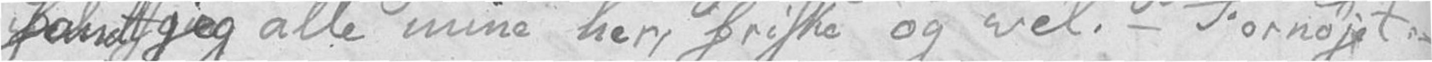fandt jeg alle mine her, friske og vel. – Fornøjet.
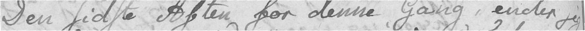Den sidste Aften for denne Gang,  ender jeg
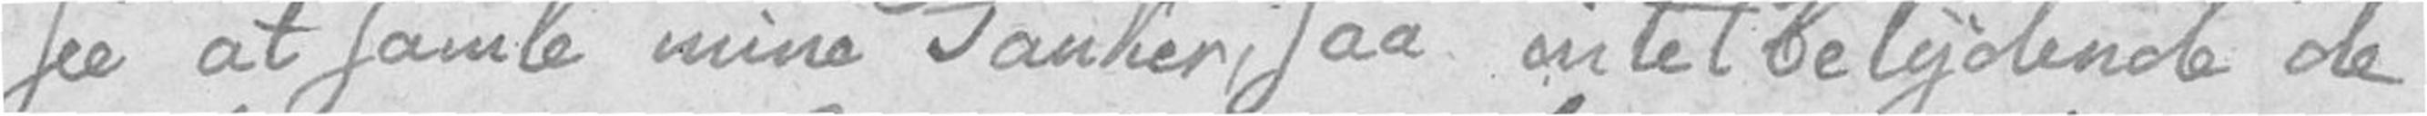see at samle mine Tanker, saa intetbetydende de
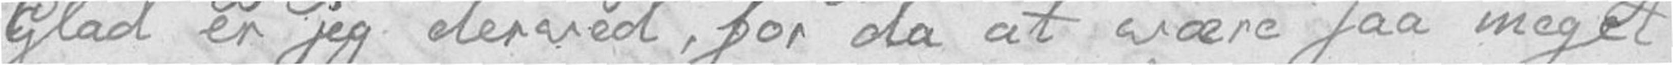Glad er jeg derved, for da at være saa meget
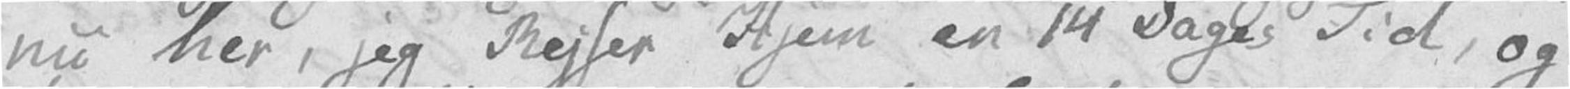nu her, jeg Rejser Hjem en 14 Dages Tid, og
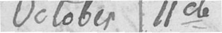October 11de
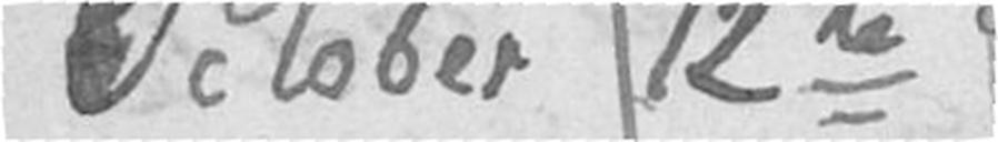October 12te
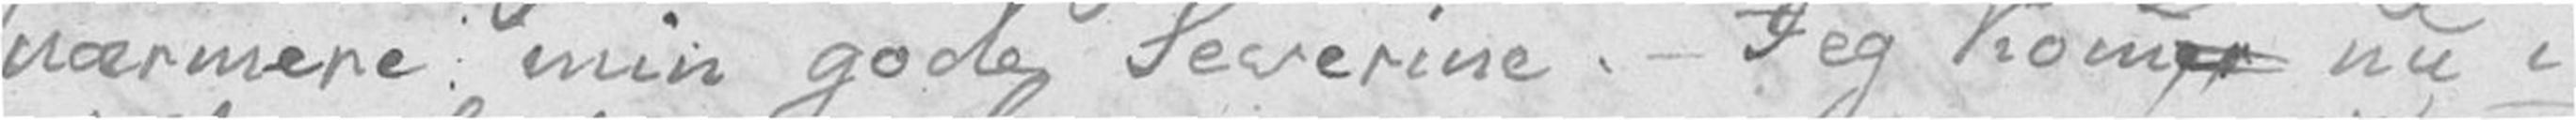nærmere min gode Severine. – Jeg komer nu i
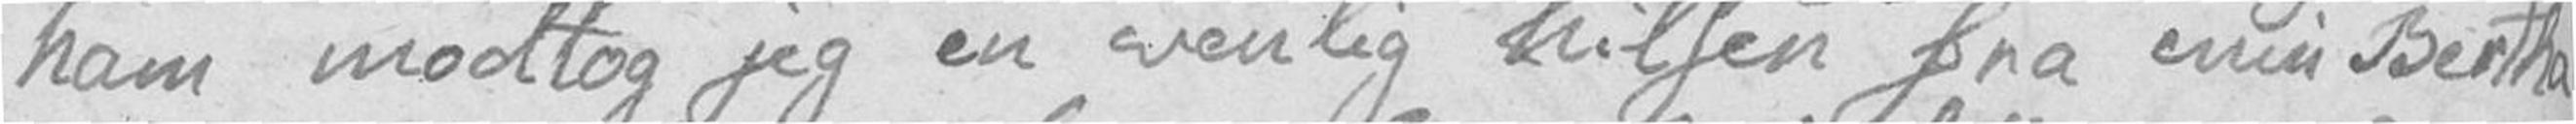ham modtog jeg en venlig hilsen fra min Bertha
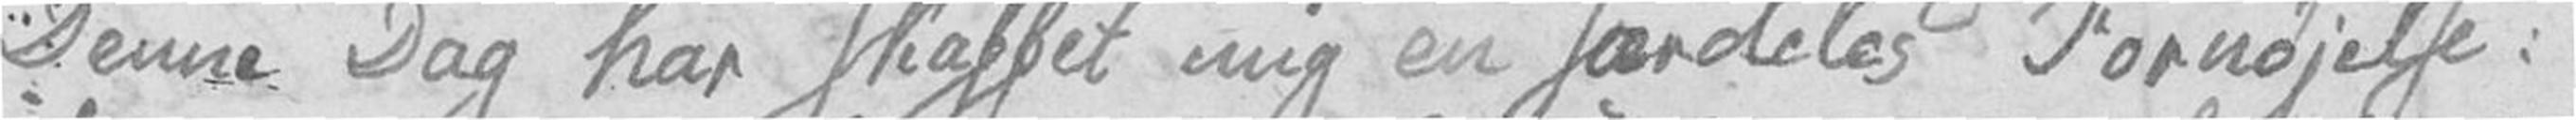Denne Dag har skaffet mig en særdeles Fornøjelse:
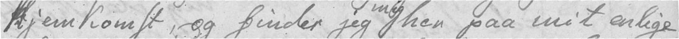hjemkomst, og finder jeg mig her paa mit enlige
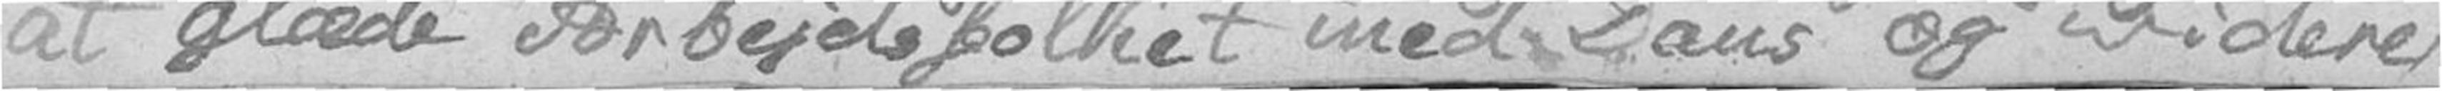at glæde Arbejdsfolket med Dans og videre,
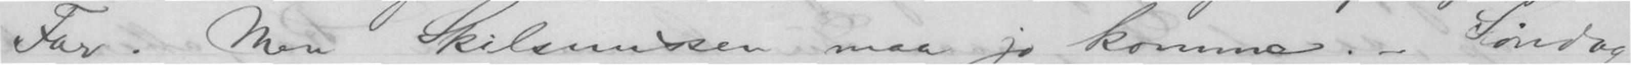Far. Men Skilsmissen maa jo komme. - Søndag
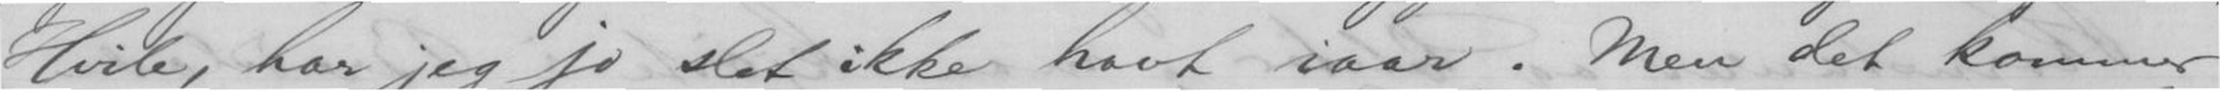Hvile, har jeg jo slet ikke havt iaar. Men det kommer
